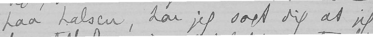paa halsen, har jeg sagt dig at jeg
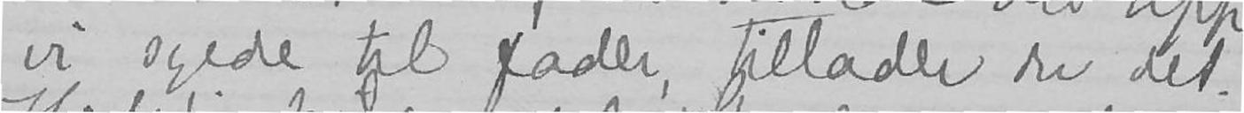vi syede til fader, tillader du det.
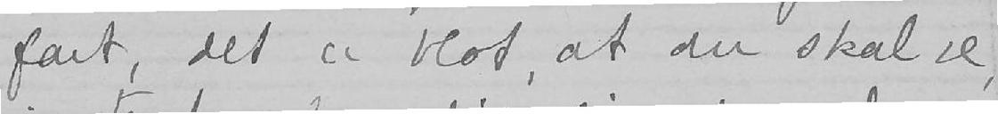fart, det er blot, at du skal se,
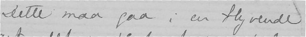Dette maa gaa i en flyvende
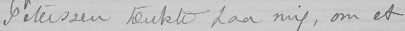Peterssen tænkte paa mig, om et
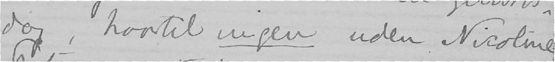dag, hvortil ingen uden Nicoline
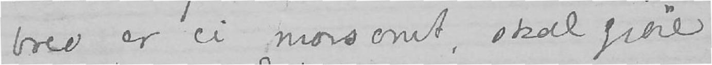brev er ei morsomt, skal gjøre
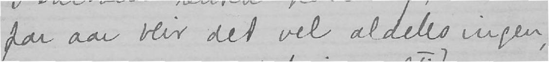par aar blir det vel aldeles ingen,
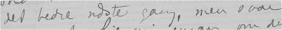det bedre næste gang, men svar
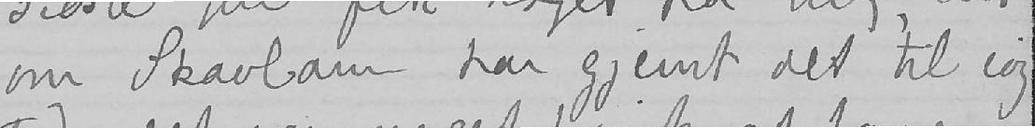om Skavlan har gjemt det til lig
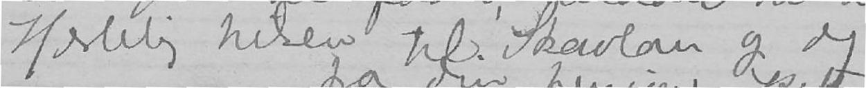Hjertelig hilsen til Skavlan og dig
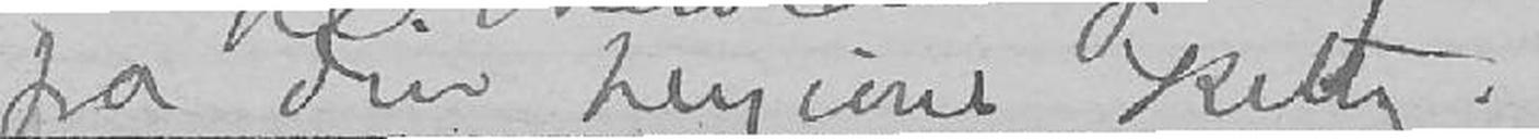fra din hengivne Kitty.
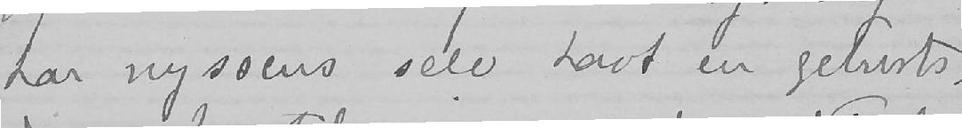har nyssens selv havt en geburts-
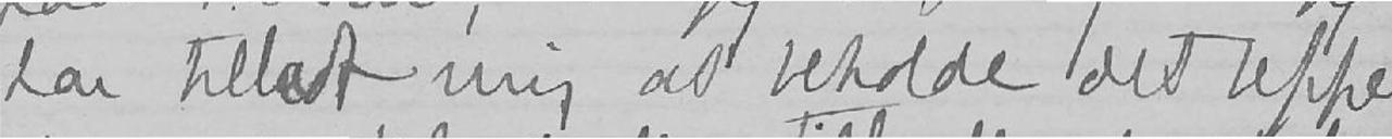har tilladt mig at beholde det teppe
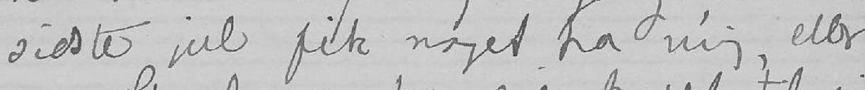sidste jul fik noget fra mig, eller
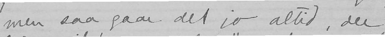men saa gaar det jo altid, der
